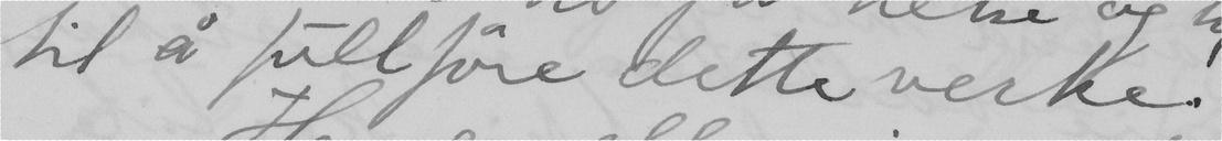til å fullföre dette verke!
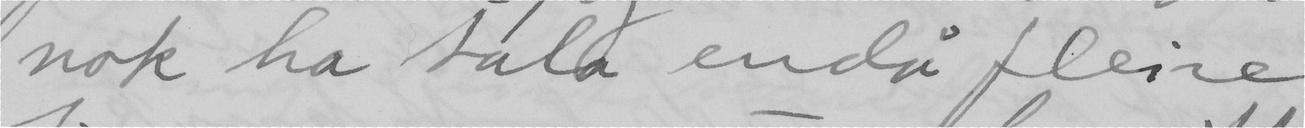nok ha tala endå fleire
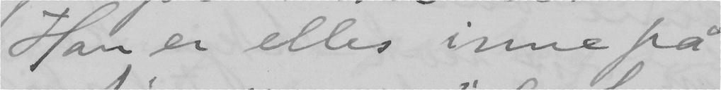Han er elles inne på
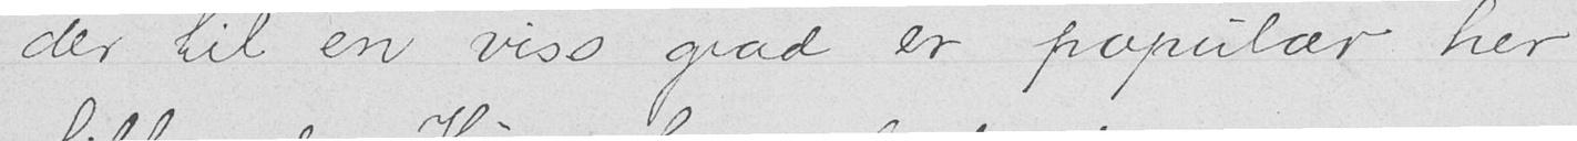der til en viss grad er populær her
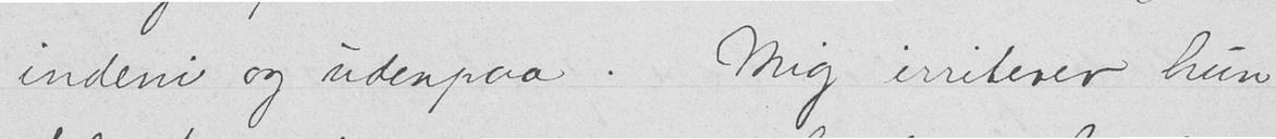indeni og udenpaa. Mig irriterer hun
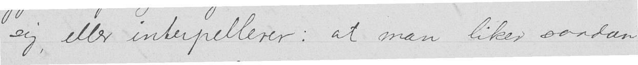sig, eller interpellerer: at man liker saadan
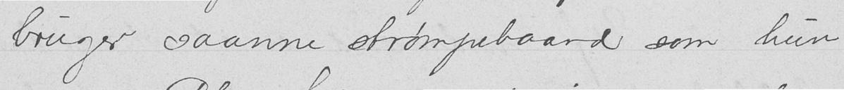bruger saanne strømpebaand som hun
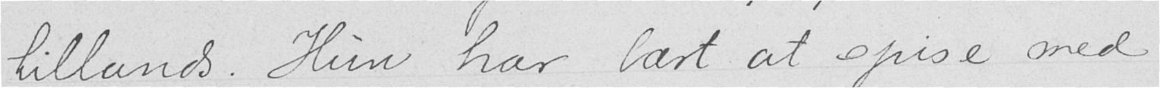tillands. Hun har lært at spise med
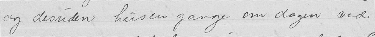og desuden tusen gange om dagen ved
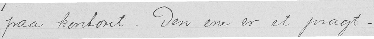paa kontoret. Den ene er et pragt-
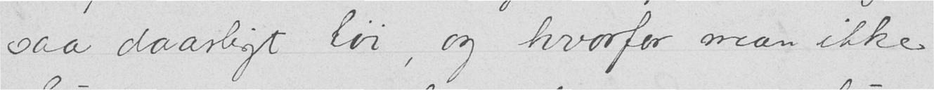saa daarligt tøi, og hvorfor man ikke
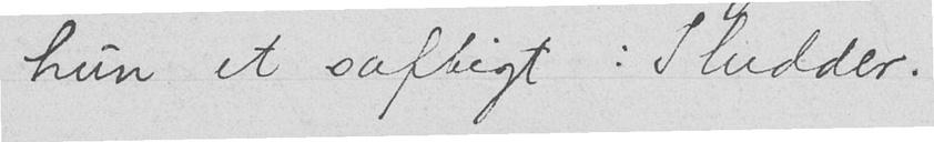hun et saftigt: Sludder!
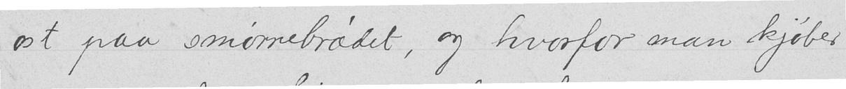ost paa smørrebrødet, og hvorfor man kjøber
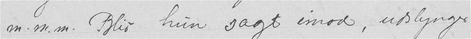m.m.m. Blir hun sagt imod, udslynger
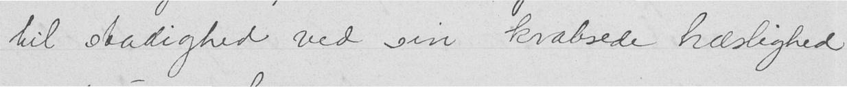til stadighed ved sin kvabsede hæslighed
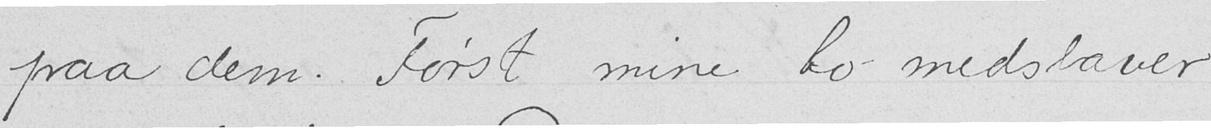paa dem. Først mine to medslaver
